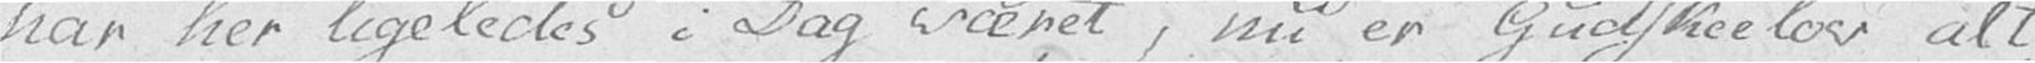har her ligeledes i Dag været, nu er Gudskelov alt
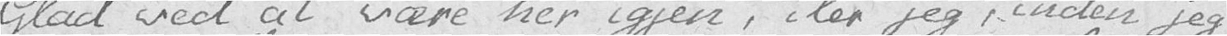Glad ved at være her igjen, iler jeg, inden jeg
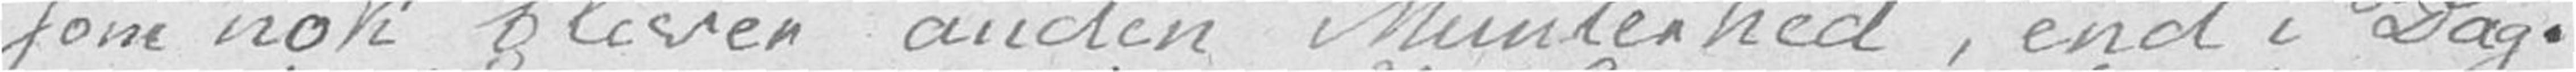som nok bliver anden Munterhed, end i Dag.
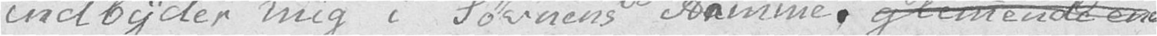indbyder mig i Søvnens Armme. glemmende end
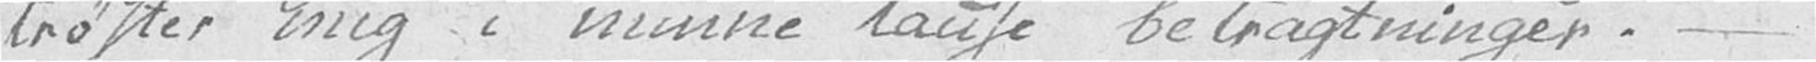trøster mig i minne tause betragtninger. –
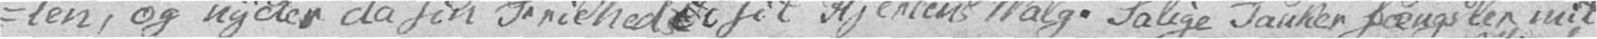-ten, og nyder da sin Friehed  i sit Hjertens Valg. Salige Tanker fængsler mit
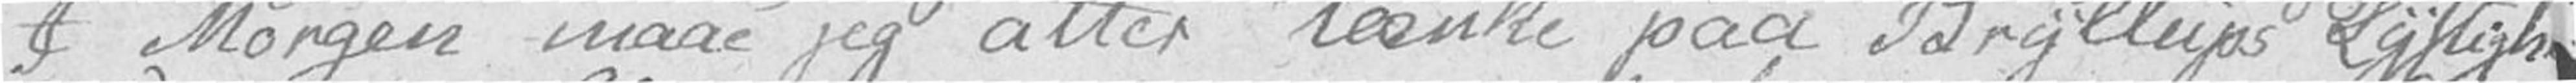I Morgen maae jeg atter tænke paa Bryllups Lystigh
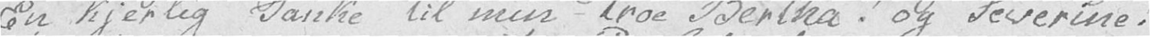En kjerlig Tanke til min troe Bertha! og Severine!
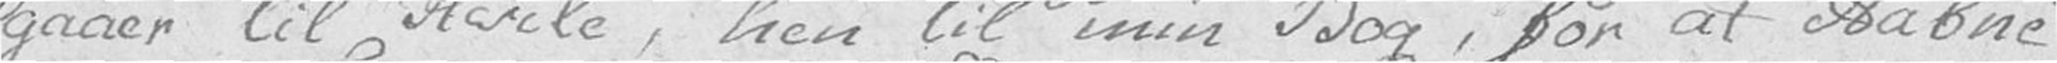gaaer til Hvile, hen til min Bog, for at Aabne
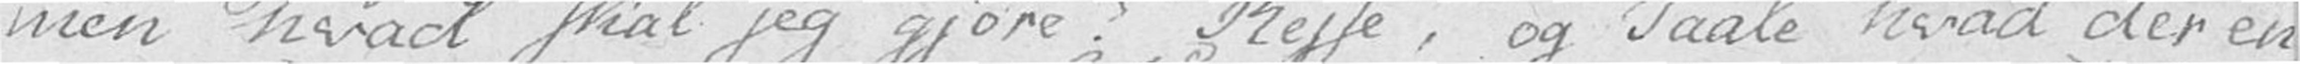men hvad skal jeg gjøre? Reise, og Taale hvad der en
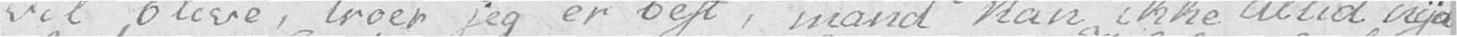vil blive, troer jeg er best, mand kan ikke altid nyd
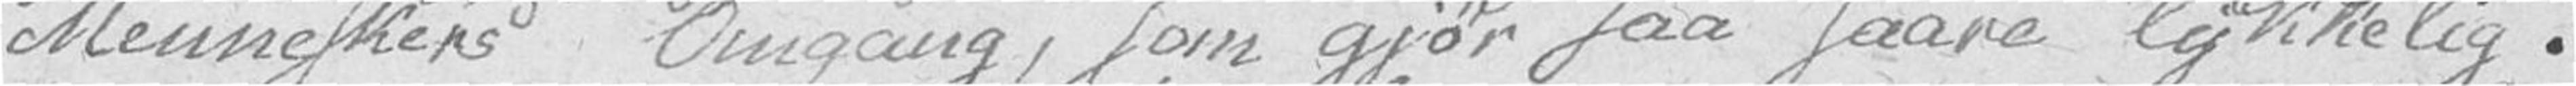Menneskers Omgang, som gjør saa saare lykkelig.
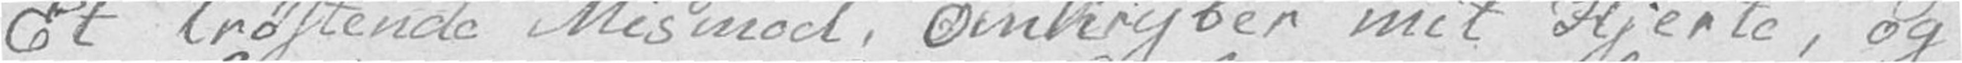Et trøstende Mismod, omkryber mit Hjerte, og
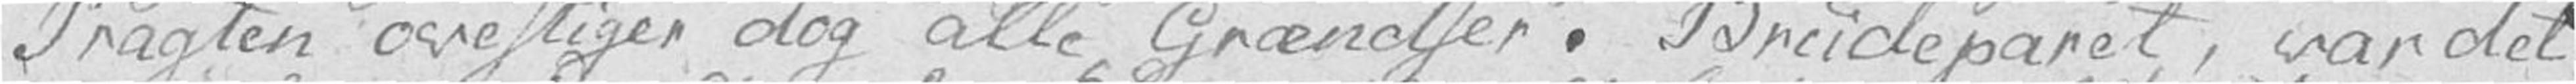Pragten overstiger dog alle Grændser. Brudeparet, var det
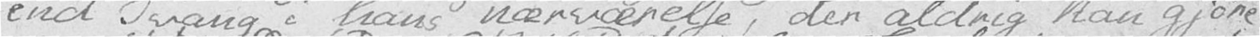end Tvang i hans nærværelse, der aldrig kan gjøre
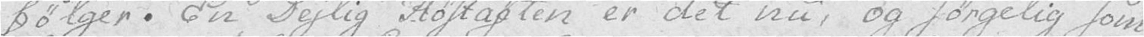følger. En Dejlig Høstaften er det nu, og sørgelig som
